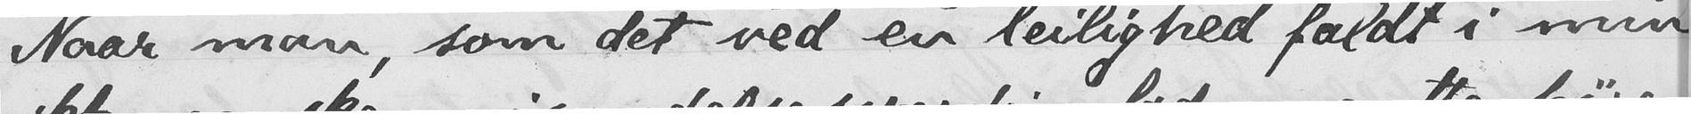Naar man, som det ved en leilighed faldt i min
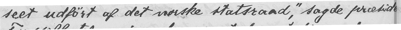seet udført af det norske statsraad", sagde præsident
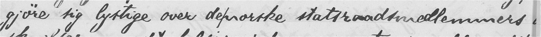gjøre sig lystige over de norske statsraadsmedlemmers an-
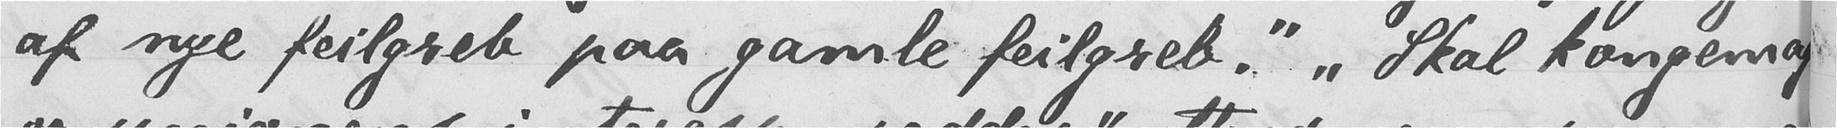af nye feilgreb paa gamle feilgreb." "Skal kongemagtens
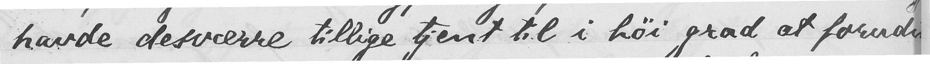havde desværre tillige tjent til i høi grad at forudin-
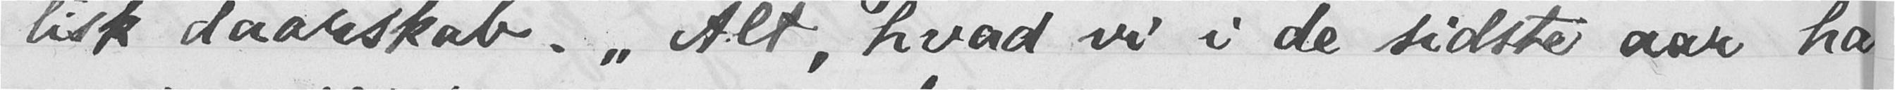tisk daarskab. "Alt, hvad vi i de sidste aar har
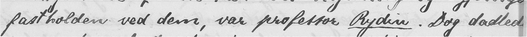fastholden ved dem, var professor Rydin. Dog dadlede
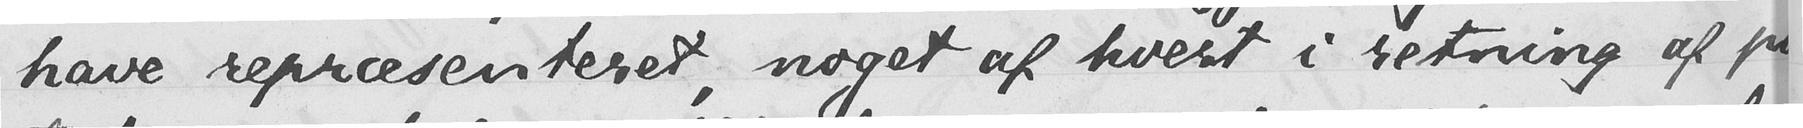have repræsenteret, noget af hvert i retning af poli-
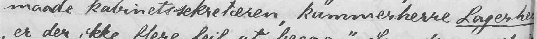maade kabinetssekretæren, kammerherre Lagerheim,
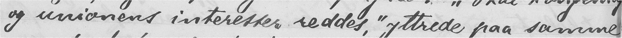og unionens interesser reddes," yttrede paa samme
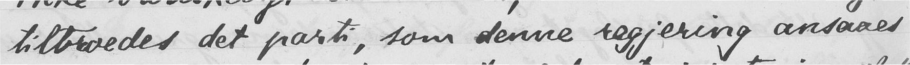tiltroedes det parti, som denne regjering ansaaes at
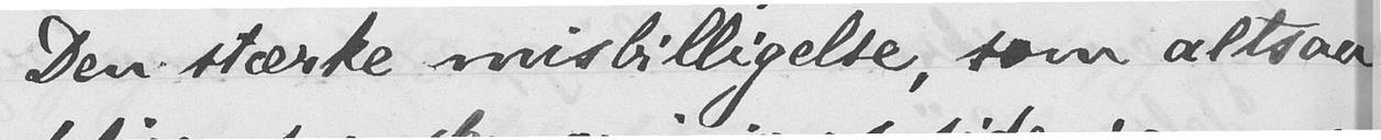Den stærke misbilligelse, som altsaa
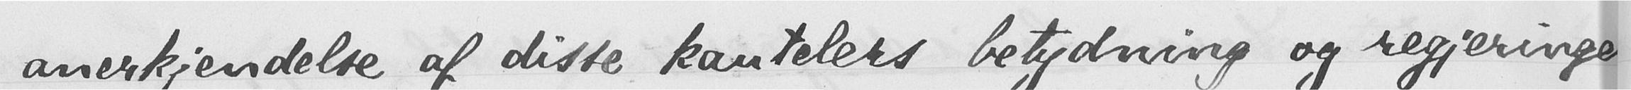anerkjendelse af disse kantelers betydning og regjeringens
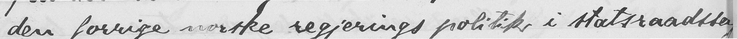den forrige norske regjerings politik i statsraadssagen
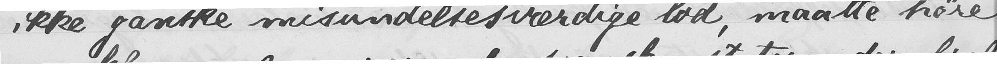ikke ganske misundelsesværdige lod, maatte høre
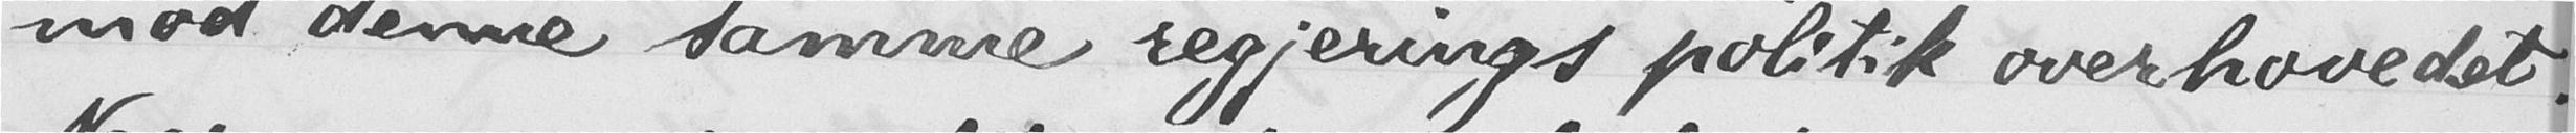mod denne samme regjerings politik overhovedet.
